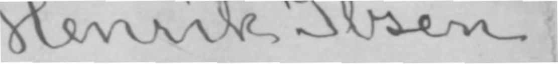Henrik Ibsen.
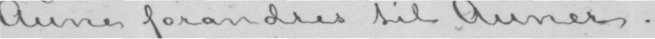Aune forandres til Auner.
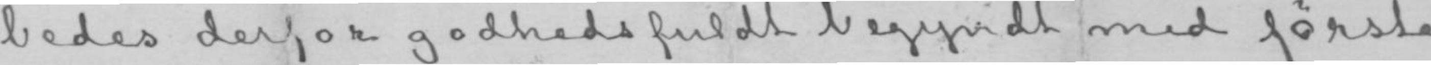bedes derfor godhedsfuldt begyndt med første
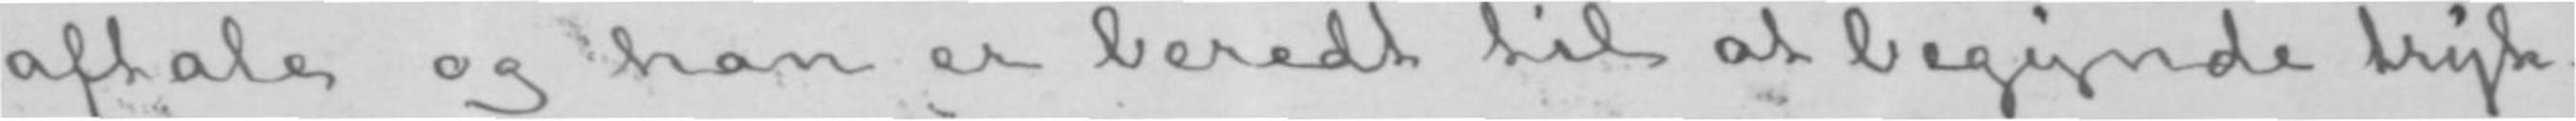aftale og han er beredt til at begynde tryk-
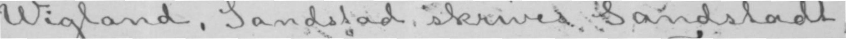Wigland, Sandstad skrives Sandstadt,
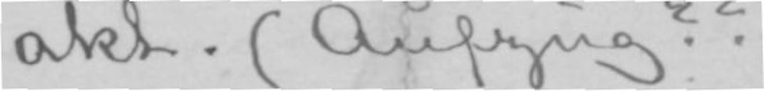akt. (Aufzug??)
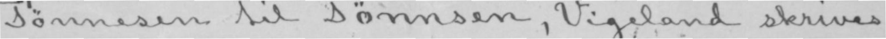Tønnesen til Tönnsen, Vigeland skrives
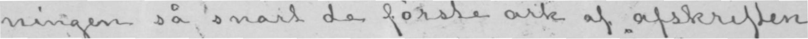ningen så snart de første ark af afskriften
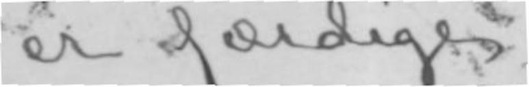er færdige.
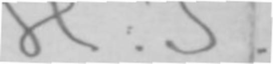H. I.
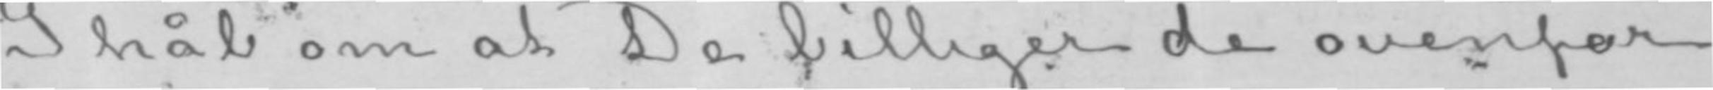I håb om at De billiger de ovenfor
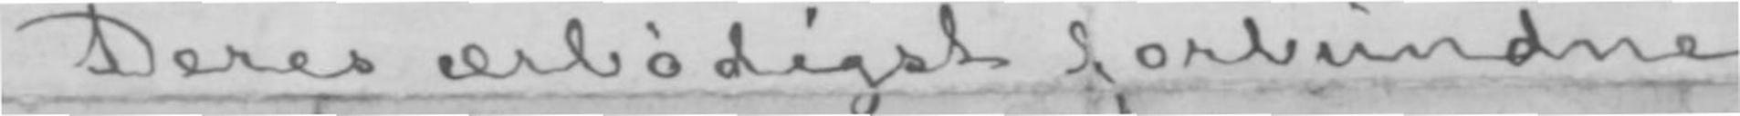Deres ærbødigst forbundne
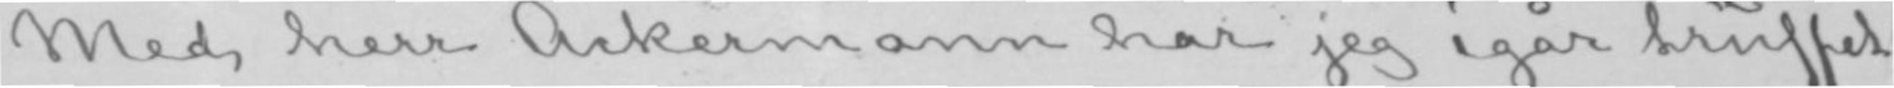Med herr Ackermann har jeg igår truffet
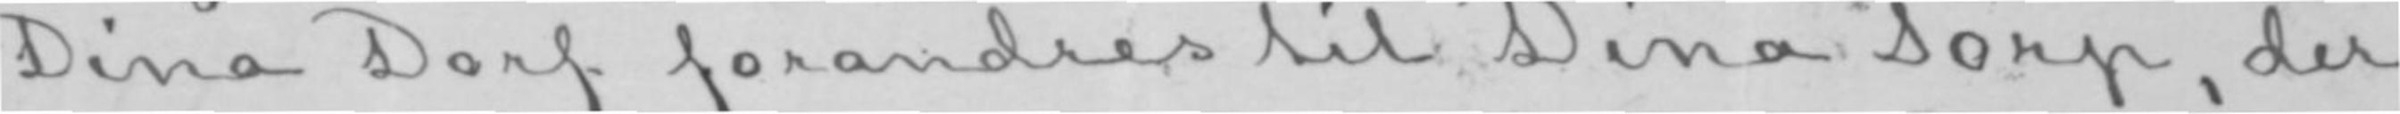Dina Dorf forandres til Dina Torp, der
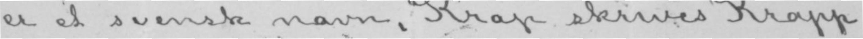er et svensk navn, Krap skrives Krapp,
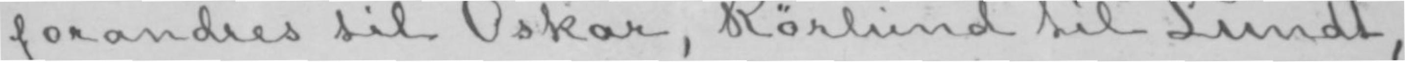forandres til Oskar, Rørlund til Lundt,
